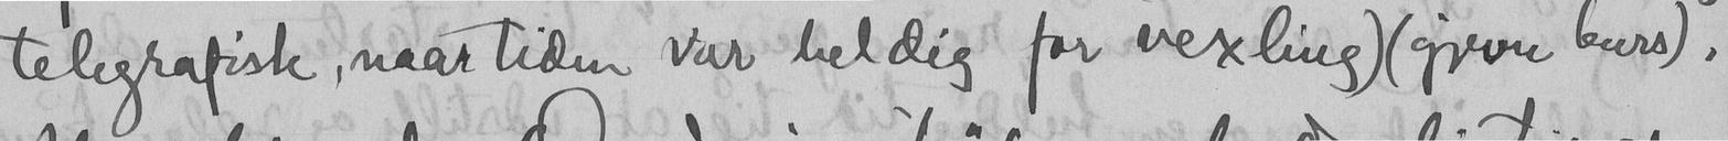telegrafisk, naar tiden var heldig for vexling) (gjevn kurs).
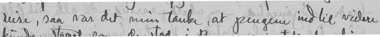reise, saa var det min tanke, at pengene, indtil videre
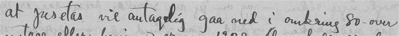at pesetas vil antagelig gaa ned i omkring 80- over
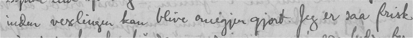inden vexlingen kan blive omigjen gjort. Jeg er saa frisk
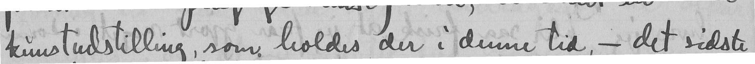kunstudstilling, som holdes der i denne tid, - det sidste
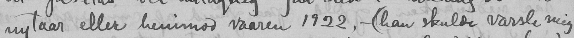nytaar eller henimod vaaren 1922, - (han skulde varsle mig
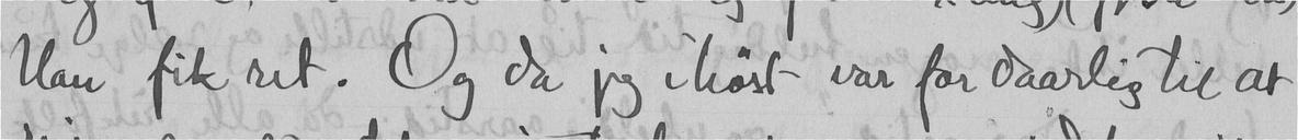Han fik ret. Og da jeg i høst var for daarlig til at
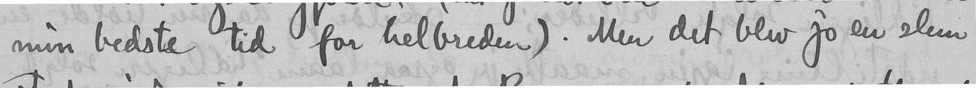min bedste tid for helbreden). Men det blev jo en slem
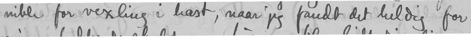nible for vexling i hast, naar jeg fandt det heldig for
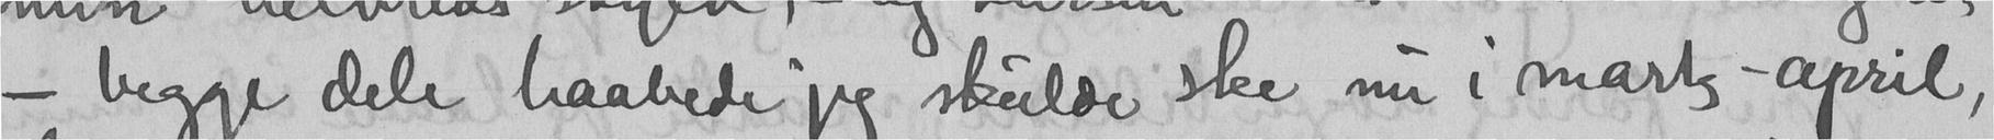- begge dele haabede jeg skulde ske nu i mars-april,
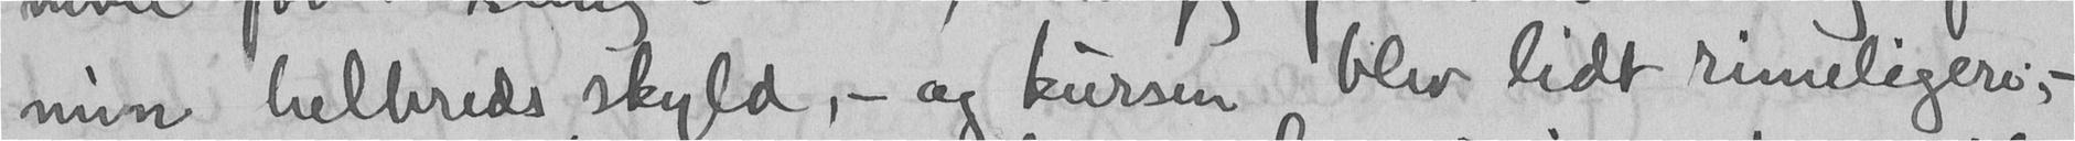min helbreds skyld, - og kursen blev lidt rimeligere; -
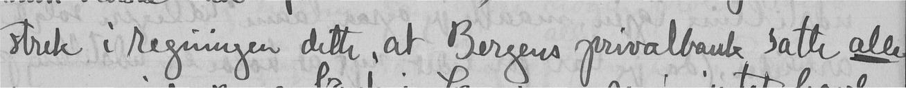strik i regningen dette, at Bergens privalbank satte alle
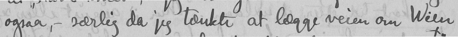ogsaa, - særlig da jeg tænkte at lægge veien om Wien
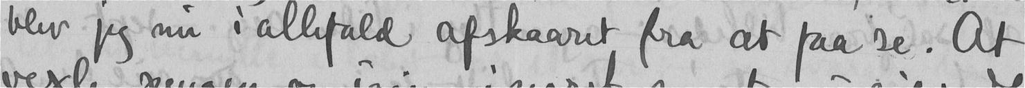blev jeg nu i allefald afskaaret fra at faa se. At
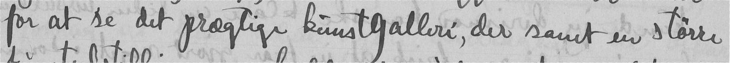for at se det prægtige kunstgalleri, der samt en større
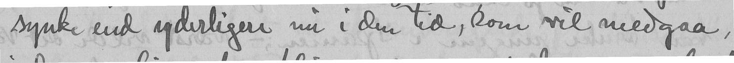synke end yderligere nu i den tid, som vil medgaa,
